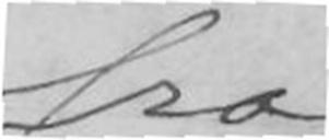fra
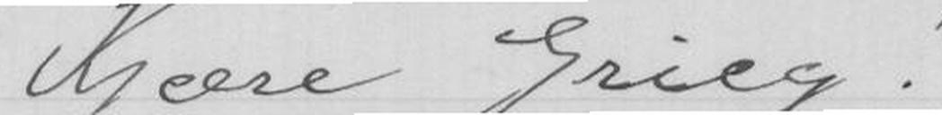Kjære Grieg!
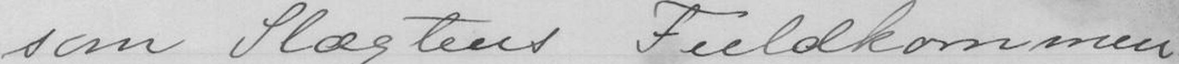som Slægtens Fuldkommen
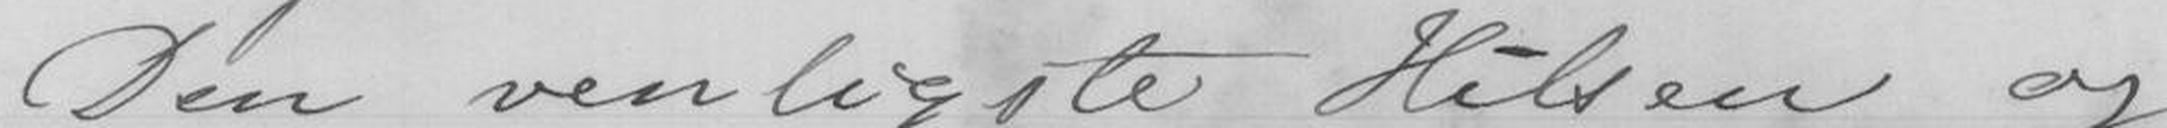Den venligste Hilsen og
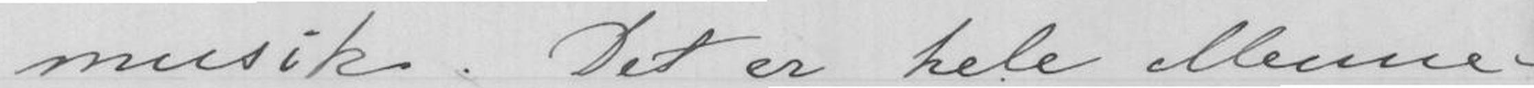musik. Det er hele Menne-
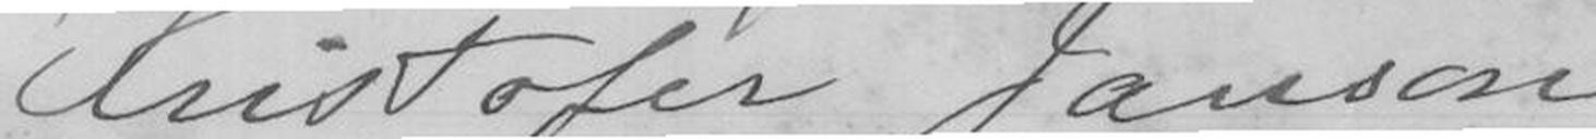Kristofer Janson
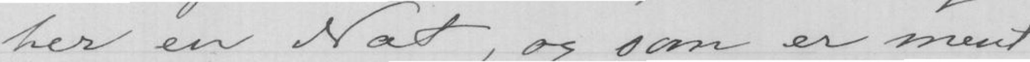her en Nat, og som er ment
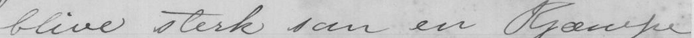blive sterk som en Kjempe
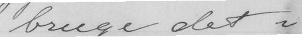bruge det?
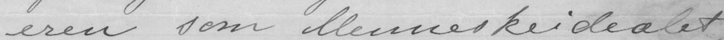eren som Menneskeidealet,
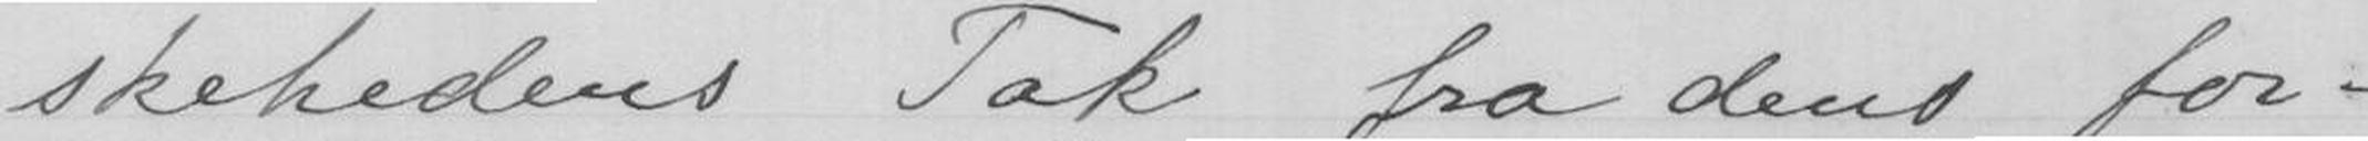skehedens Tak fra dens for-
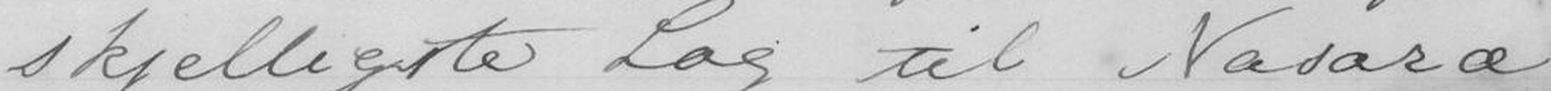skjelligste Lag til Nasaræ
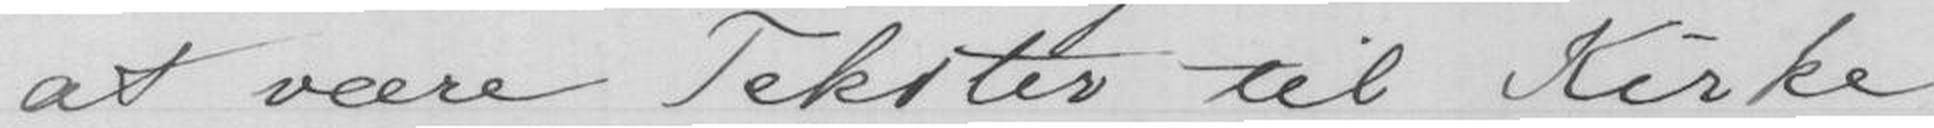at være Tekster til Kirke
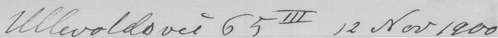Ullevoldsvei 65III 12 Nov 1900
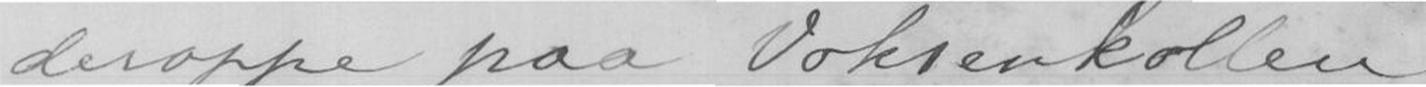deroppe paa Voksenkollen
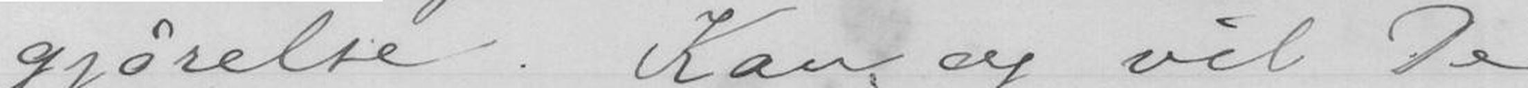gjørelse. Kan og vil De
